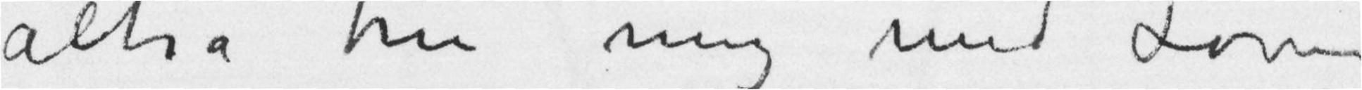altså true mig med Loven
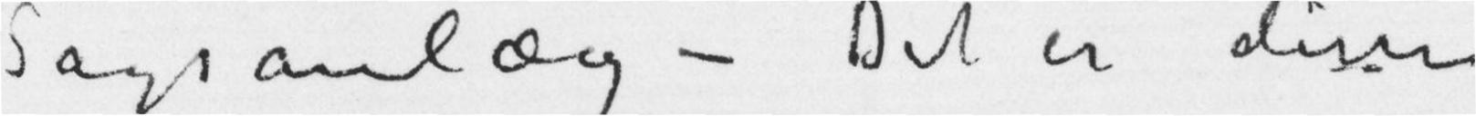Sagsanlæg – Det er disse
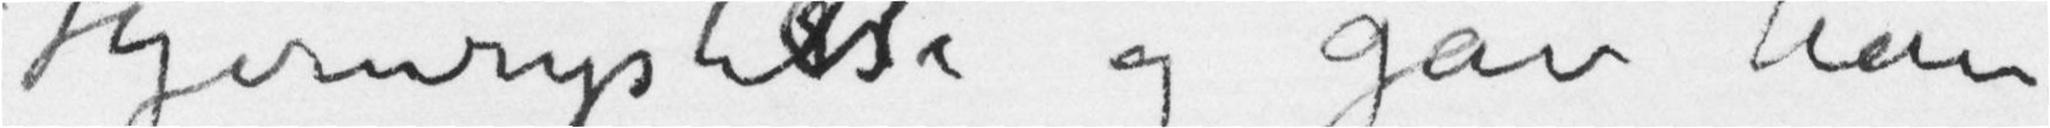Hjernerystelse og gav ham
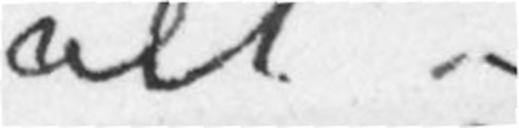alt –
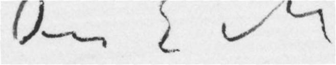Din E M
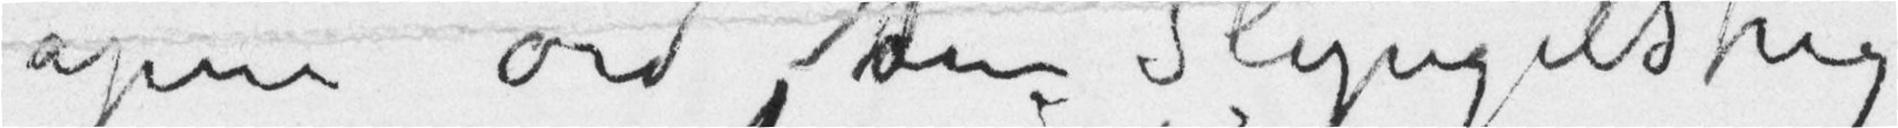åpne ord den Slyngelstreg
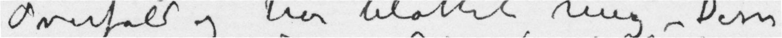Overfald og har blottet mig – Disse
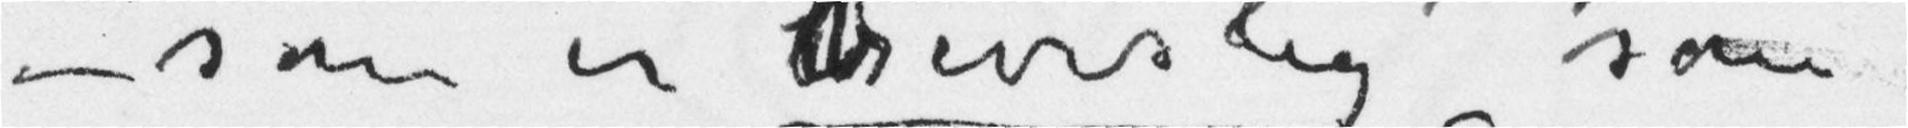– som er bevislig som
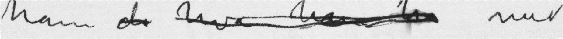ham da hva han ha med
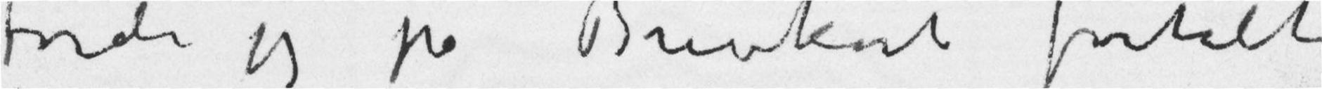fordi jeg på Brevkort fortalte
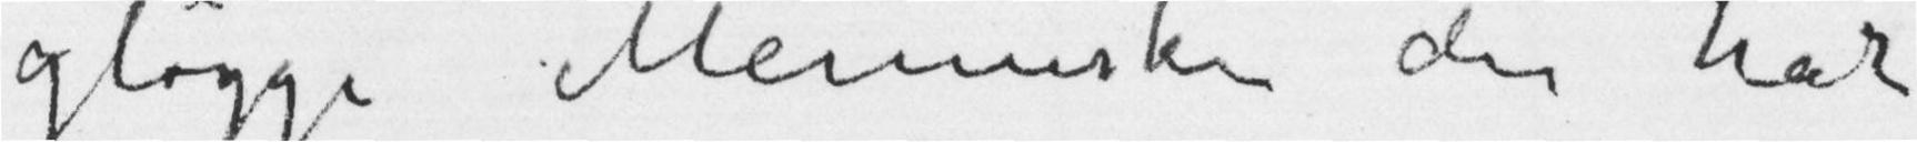gløgge Mennesker der har
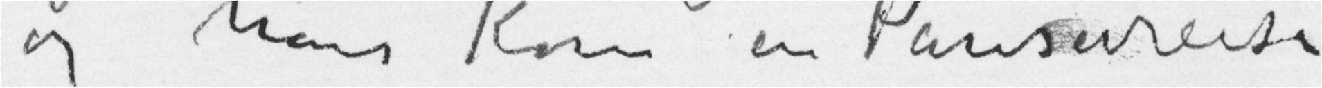og hans Kone en Pariserreise
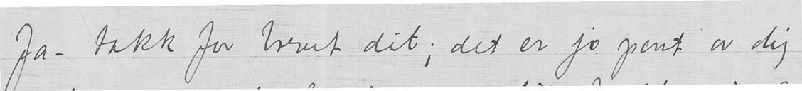Ja – takk for brevet dit; det er jo pent av dig
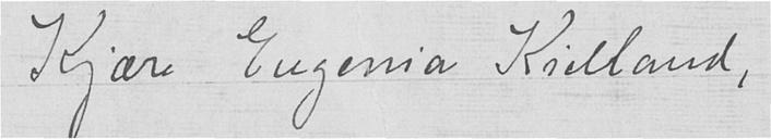Kjære Eugenia Kielland,
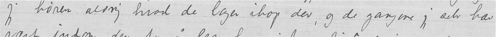jeg hører aldrig hvad de lager ihop der, og de gangene jeg selv har
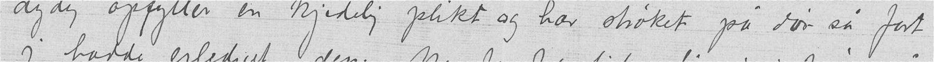dydig opfyller en kjedelig plikt og har strøket på dør så fort
